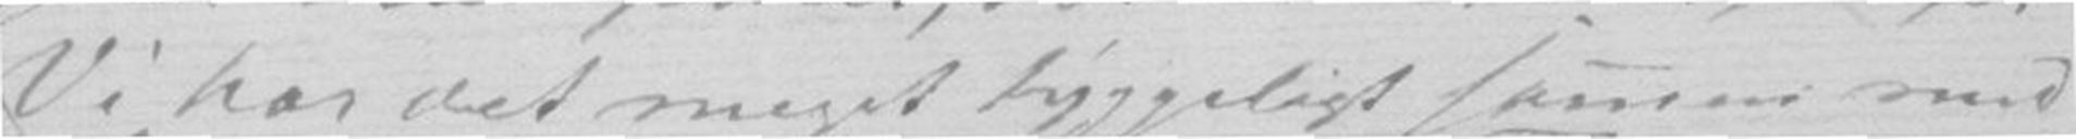Vi har det meget hyggeligt samen med
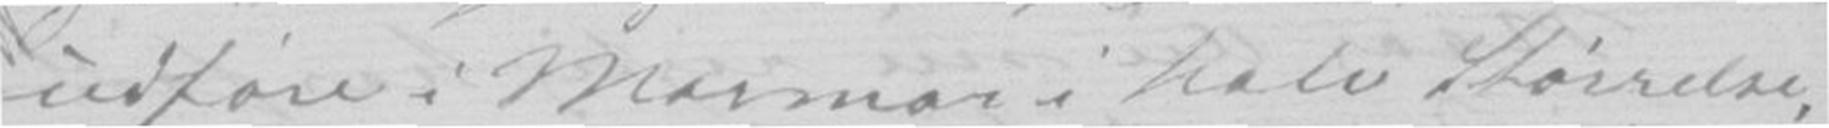udføre i Marmor i halv Størrelse,
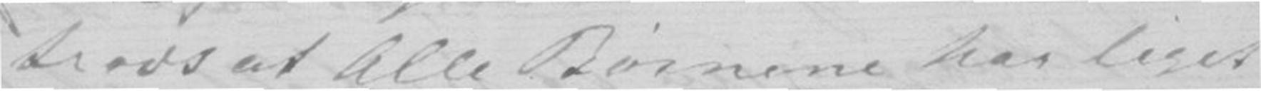trods at Alle Børnene har liget
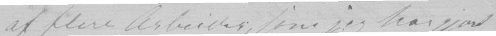af flere Arbeider, som jeg har gjort
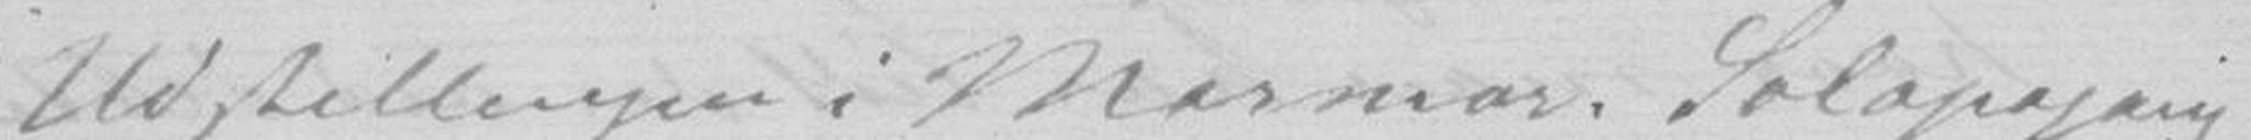Udstillingen i Marmor. Solopgang
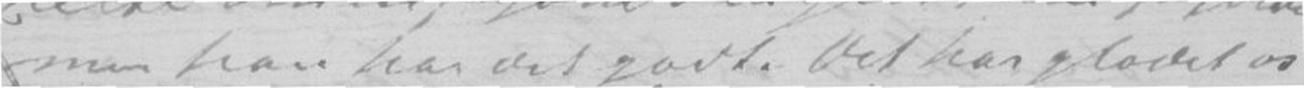men han har det godt. Det har glædet os
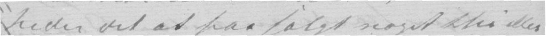heder det at faa solgt noget thi eller
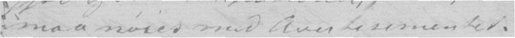maa nøies med Avertesementet.
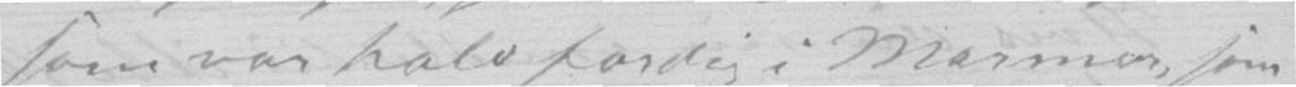som var halv færdigi Marmor som
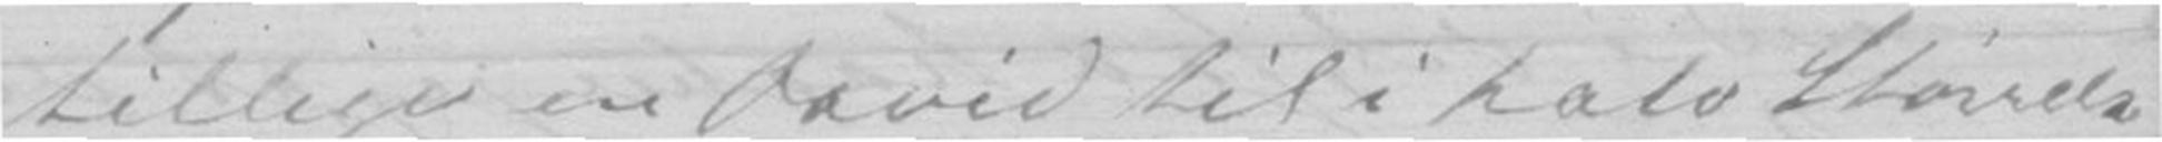tillige en David til i halv Størrelse
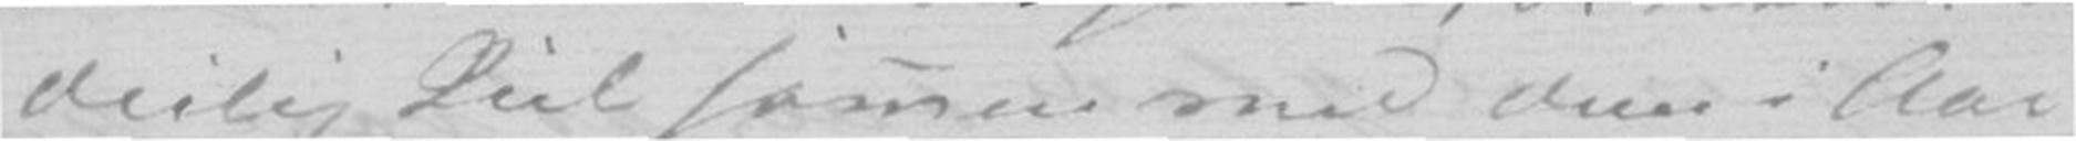deilig Jul samen med dem i Aar
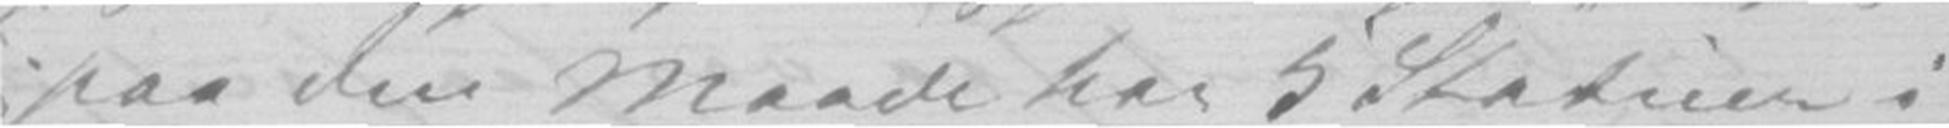paa den Maade har 5 Statuer i
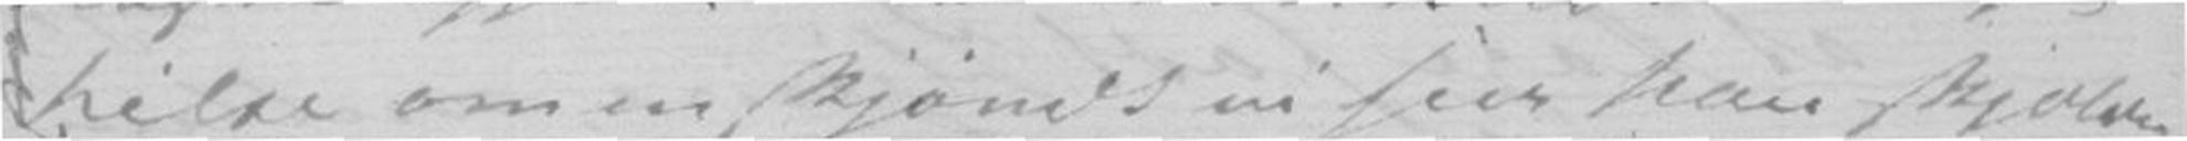hilse om en skjøndt vi seer han skjælden
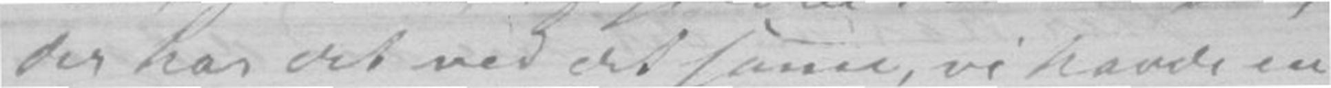der har det ved det same, vi havde en
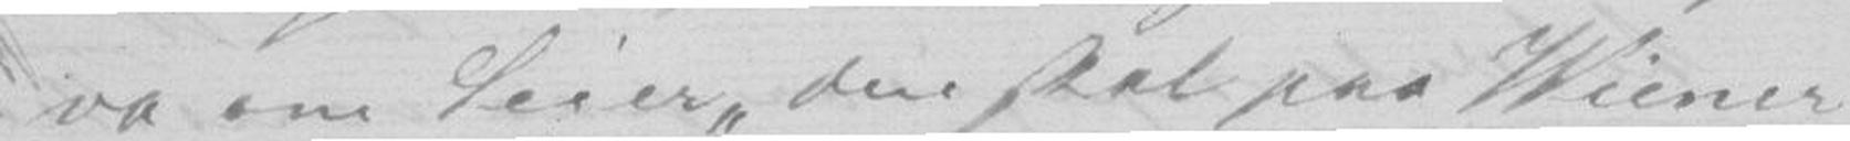va om Seier den skal paa Wiener
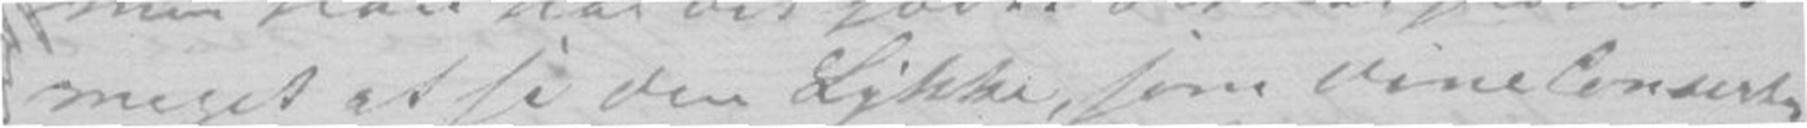meget at se den Lykke, som dine Conserter
